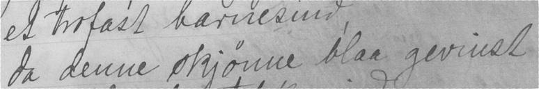da denne skjønne blaa gevinst
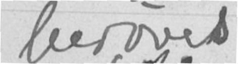berøves
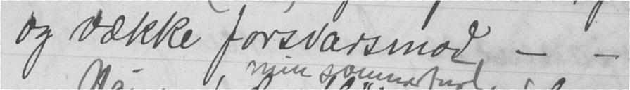og vække forsvarsmod - -
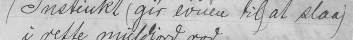Instinkt (gir evnen til) at slaa
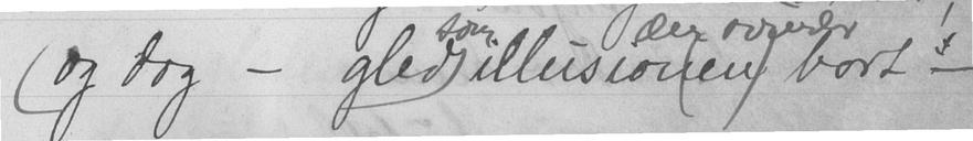(og dog - gled) som illusion(en) bort -
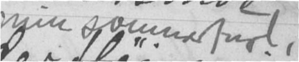min sommerfugl,
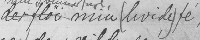der fløi min (hvide) fe,
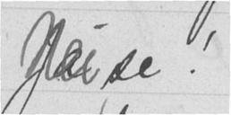Nei se!
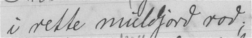i rette muldjord rod;
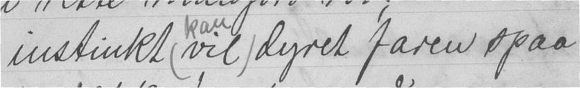instinkt (vil) kan dyret faren spaa
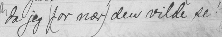da jeg (for nær) den vilde se!
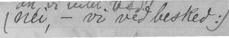(nei, - vi ved besked:)
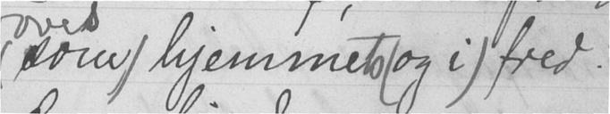(som) hjemmets (og i) fred!
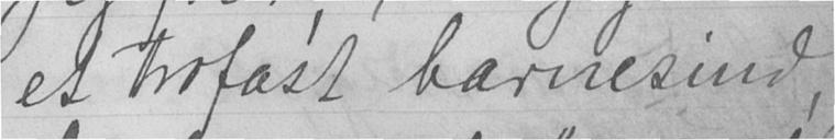et trofast barnesind,
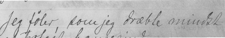Jeg føler, som jeg dræbte mindst
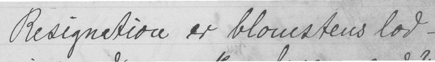Resignation er blomstens lod -
